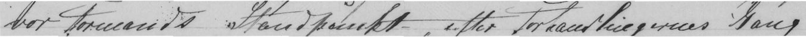vor Formands Standpunkt, efter Forhandlingernes Gang
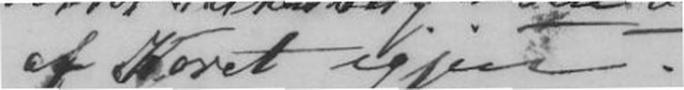af Koret igjen.
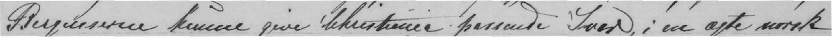Bergenserne kunne give Christiania passende Sved, i en ægte norsk
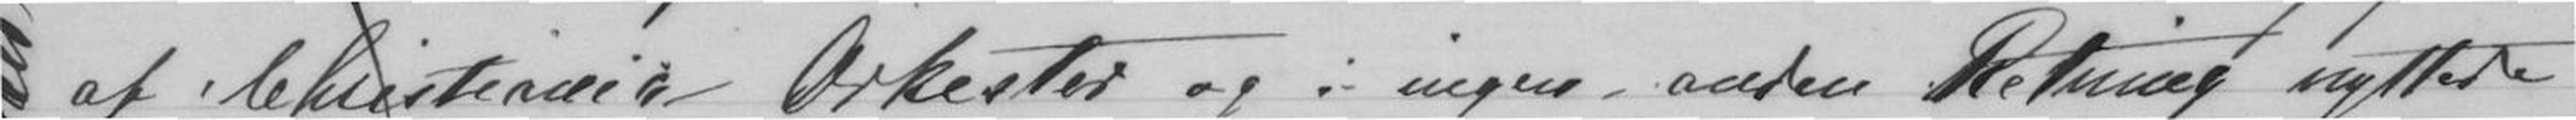af Christiania Orkester og i ingen anden Retning nytter
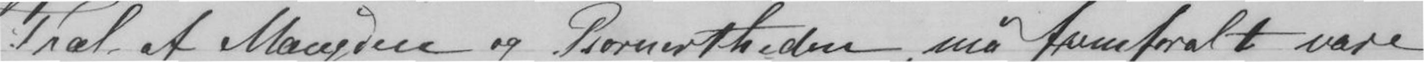Træl af Mængden og Pionerheden, må fremforalt være
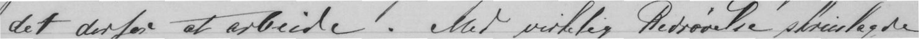det derfor at arbeide. Med virkelig Bedrøvelse skrinlagde
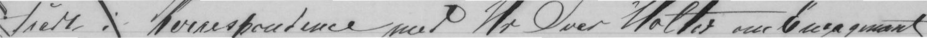trådt i Korrespondence med Hr Iver Holter om Engagement
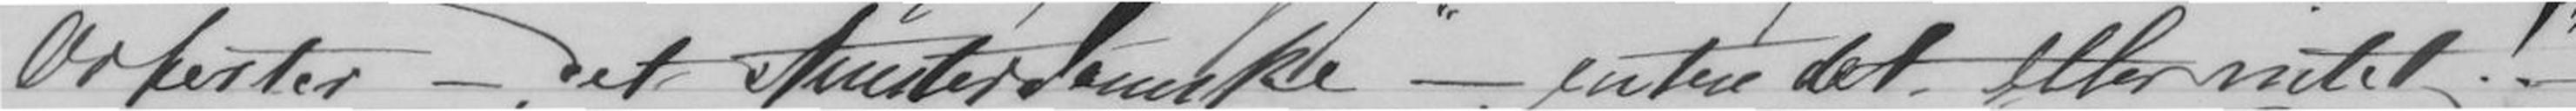Orkester - det Amsterdamske - enten det eller intet!
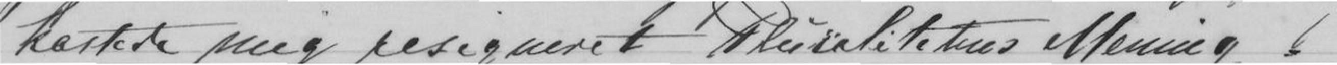kastede mig resigneret Pluralitetens Mening -
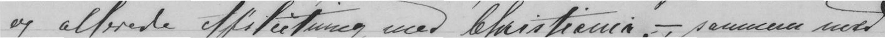og allerede Afslutning med Christiania, - sammen med
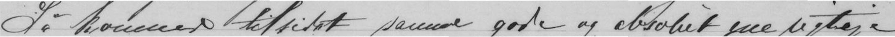Så kommer tilsidst samme gode og absolut ene rigtige
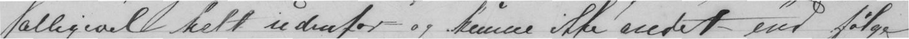alligevel helt udenfor og kunne ikke andet end følge
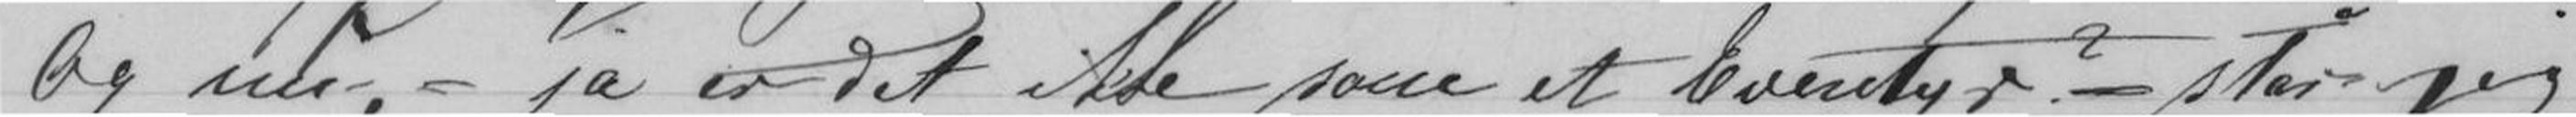Og nu, - ja er det ikke som et Eventyr? - står jeg
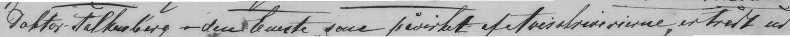Doktor Falkenberg - den Eneste som påvirket af Avisskriverierne, er trådt ud
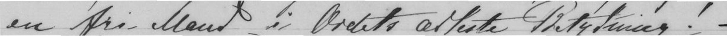en fri Mand i Ordet ædleste Betydning! -
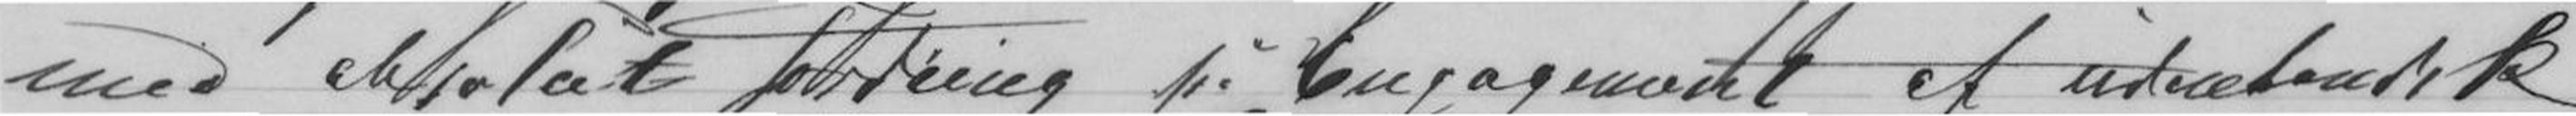med absolut fordring på Engagement af udenlandsk
